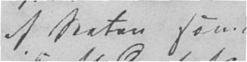af Staten som
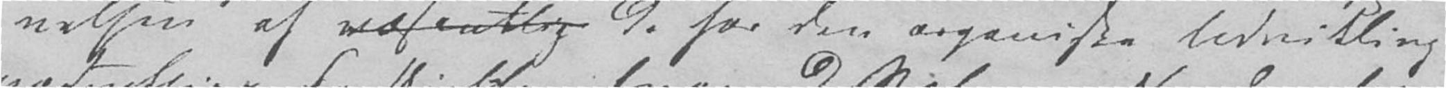nelsen af væsentlige de for den organiske Udvikling
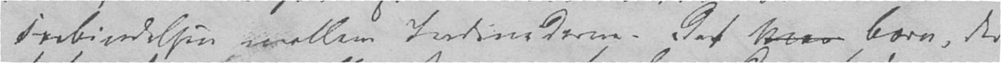Forbindelsen mellem Individerne. Det Men Børn, der
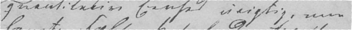qvantitativ Eenhed urigtig, men
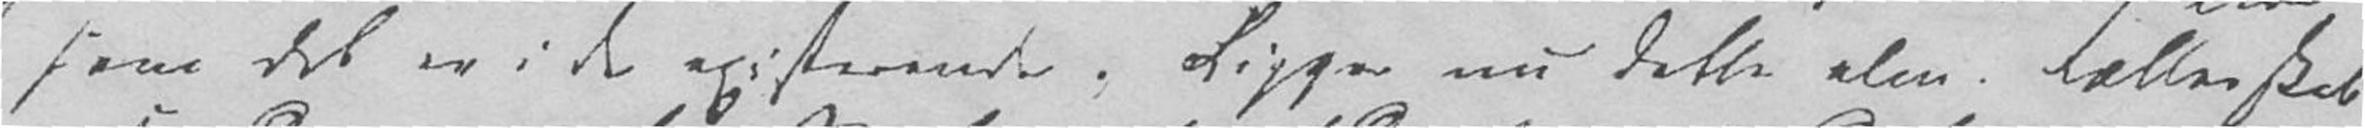som det er i de existerende. Ligger nu dette alm. Fællesskab
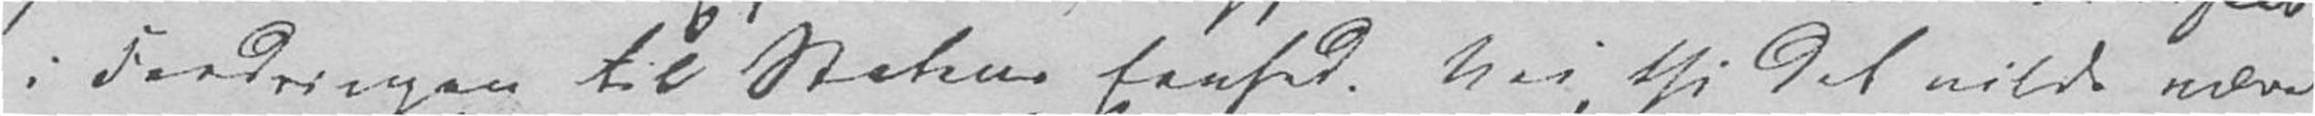i Fordringen til Statens Eenhed. Nei, thi det vilde være
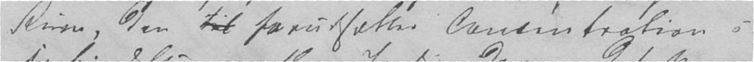Rum, den til forudsætter Concentration i
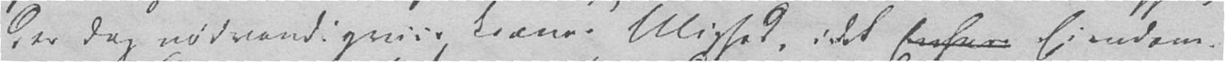der dog nødvendigviis kræver Ulighed, idet Enhver Eiendom-
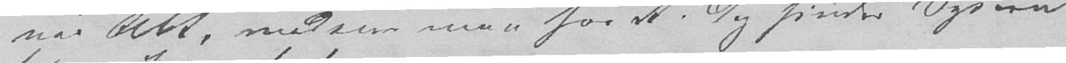var Alt, medens man hos A. dog finder Spiren
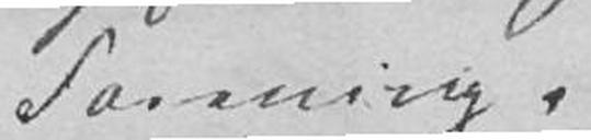Forening.
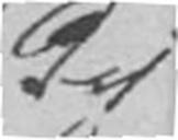det
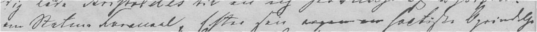om Statens Formaal. Efter sin factiske Oprindelse
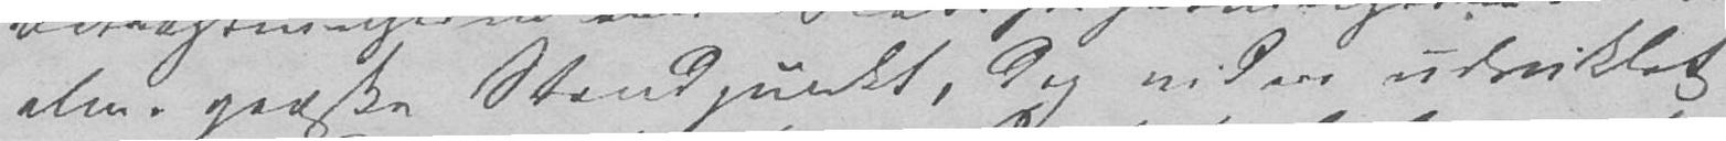alm. græske Standpunkt, dog videre udviklet
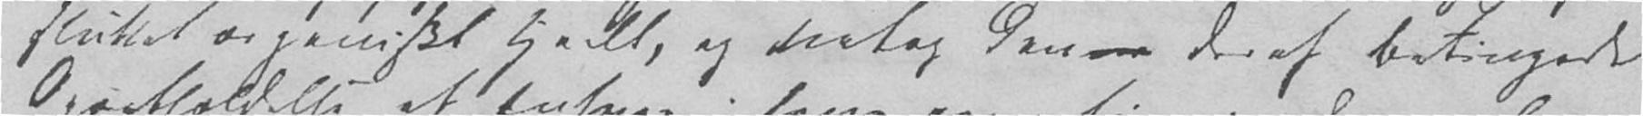sluttet organiskt Heelt, og netop denne deraf betingede
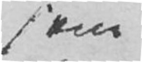som
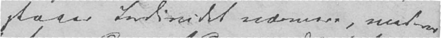staaer Individet nærmere, medens
